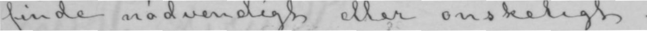finde nødvendigt eller ønskeligt.
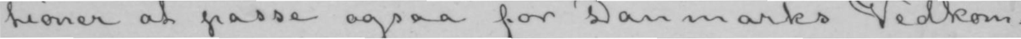tioner at passe ogsaa for Danmarks Vedkom-
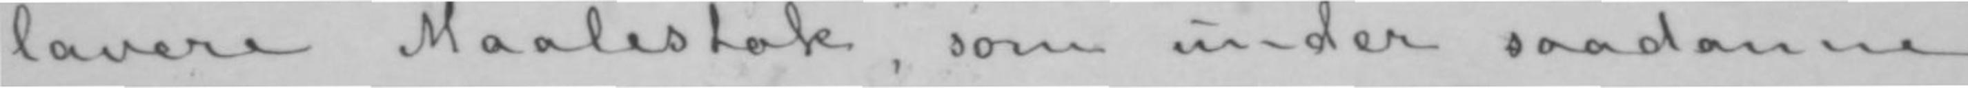lavere Maalestok, som under saadanne
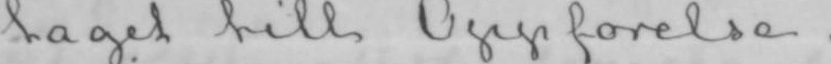taget till Oppførelse.
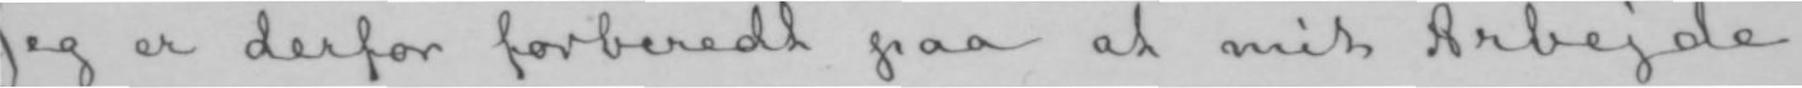Jeg er derfor forberedt paa at mit Arbejde,
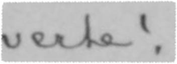verte!
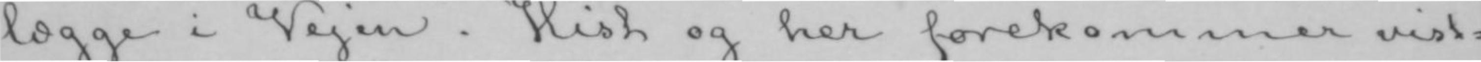lægge i Vejen. Hist og her forekommer vist-
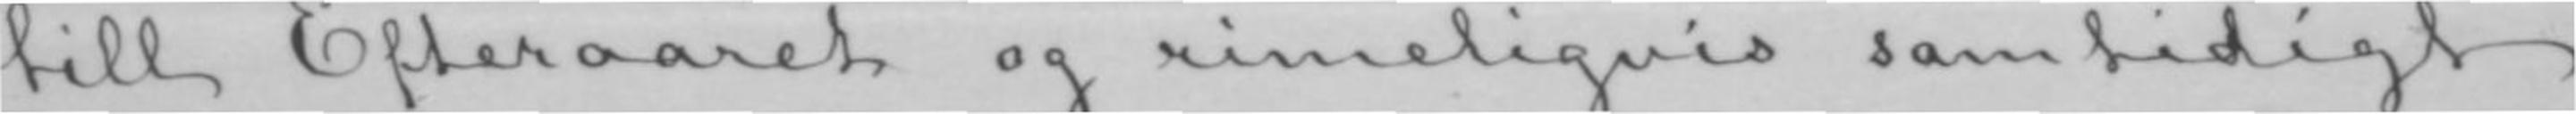till Efteraaret og rimeligvis samtidigt
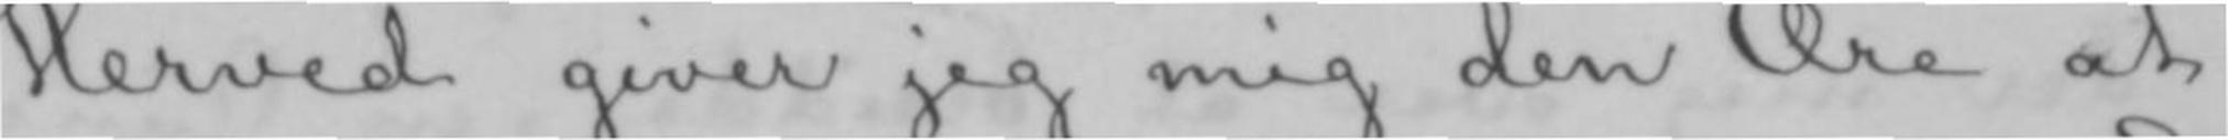Herved giver jeg mig den Ære at
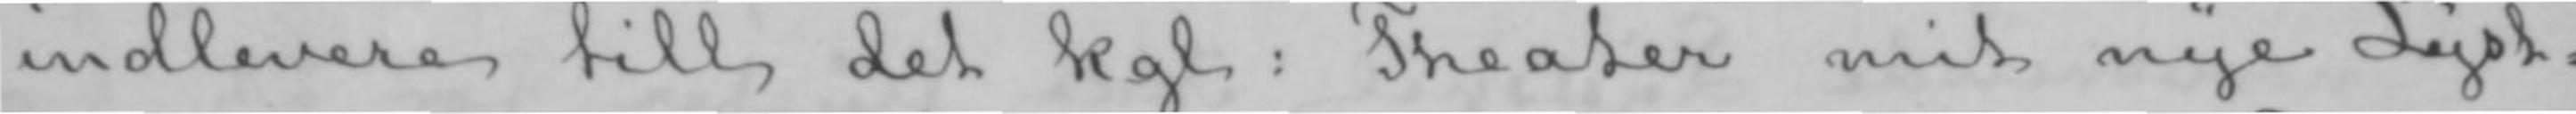indlevere till det kgl: Theater mit nye Lyst-
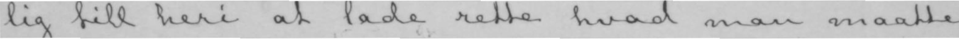lig till heri at lade rette hvad man maatte
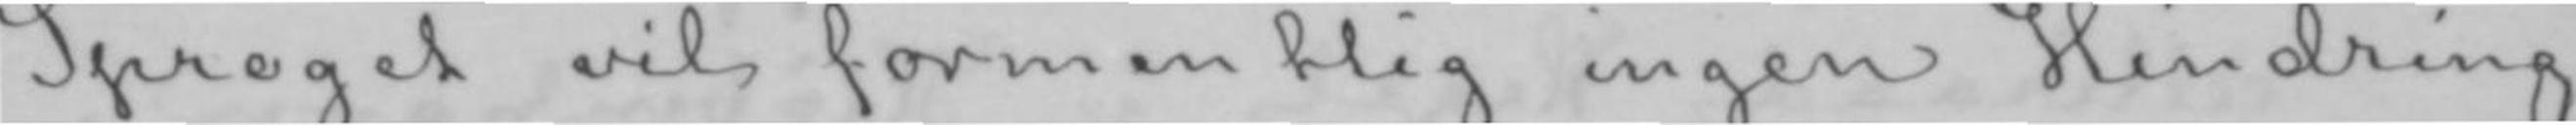Sproget vil formentlig ingen Hindring
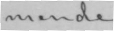mende.
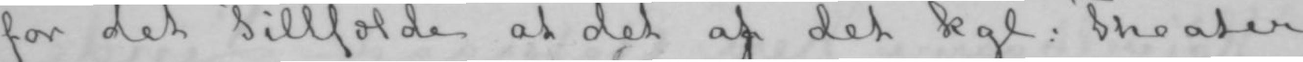for det Tillfælde at det af det kgl: Theater
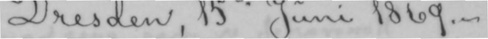Dresden, 15de Juni 1869.-
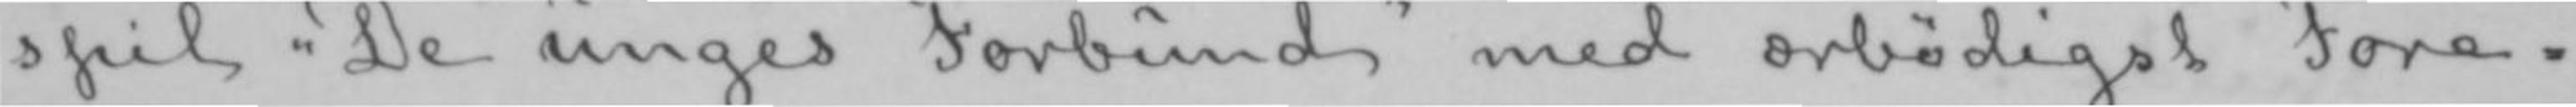spil «De unges Forbund» med ærbødigst Fore-
